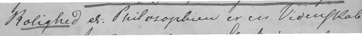Rolighed el: Philosophien er en Videnskab
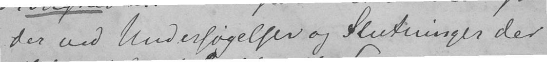der ved Undersøgelser og Slutninger der
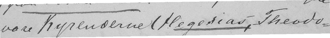vare Kyrenæerne (Hegerias, Theodo-
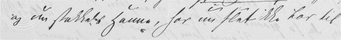og nu stakkels Hanne, har nu slet ikke Lør til
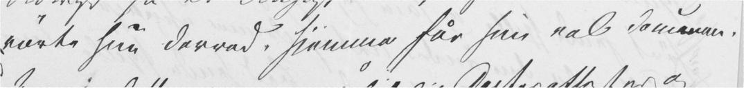rørte hun derved, hjemme får hun vel documen-
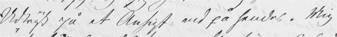Udtryk på et Ansigt end på hendes. Mig
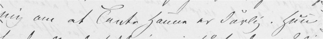mig om at Tante Hanne er darlig. Hun
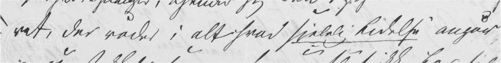ret, der råder i alt hvad sjelelig Lidelse angår
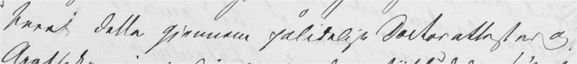teret dette gjennem pålidelige Doctorattester og
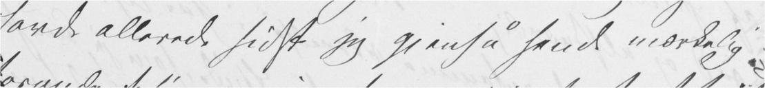havde allerede sidst jeg gjenså hende mærkelig
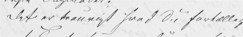Det er traurigt hvad Du fortæller,
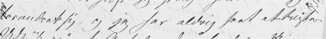forandret sig, og jeg har aldrig seet et tristere
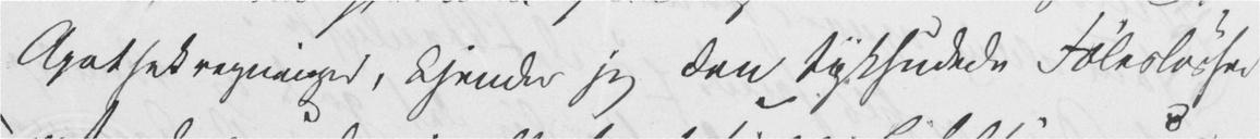Apothekregninger, kjender jeg den tykhudede Følesløshed
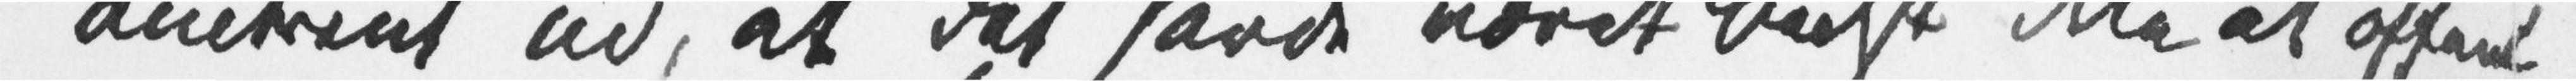omtrent ud, at det havde været bedst ikke at offent¬
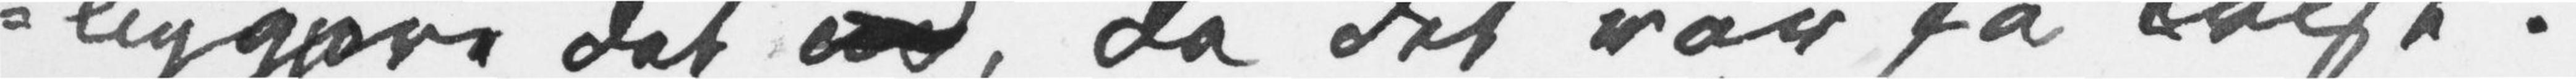liggjøre det ud, da «det var så trist».
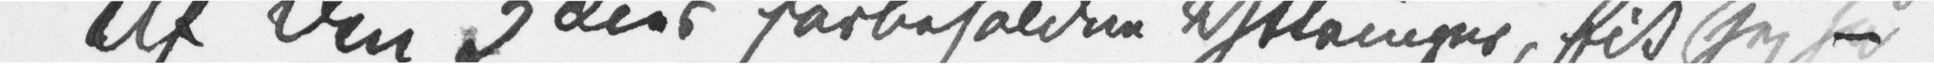Af den 3dies forbeholdne Yttringer, fik jeg så
